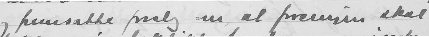og fremsatte forslag om at foreningen skal
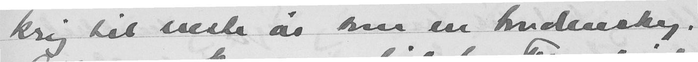krig til neste år som en tordensky.
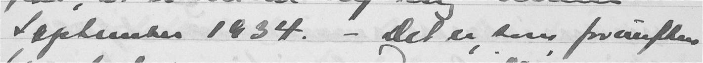September 1934. - Det er, som fornuften
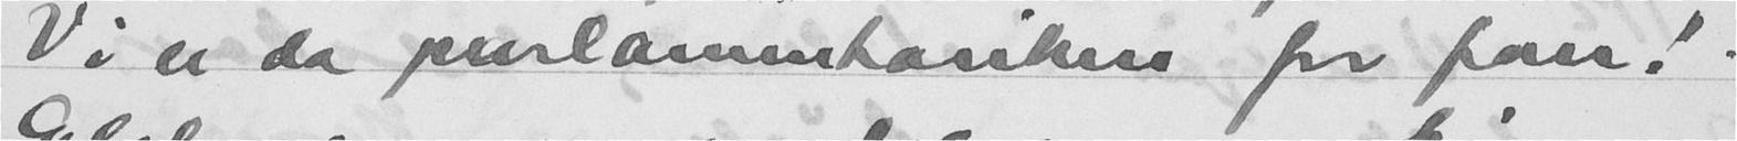Vi er da parlamentariske for fan! -
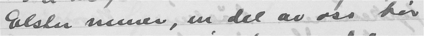Elster mener, en del av oss får
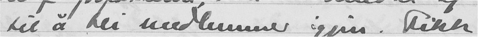til å bli medlemmer igjen. Fikk
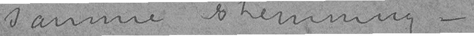samme stemning –
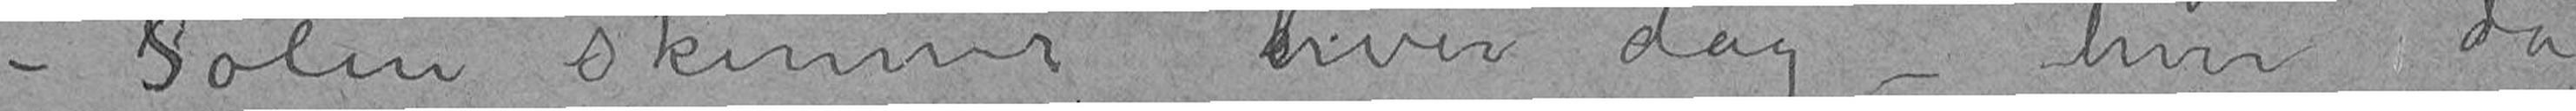– Solen skinner hver dag – hver dag
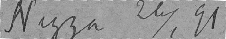Nizza 26/1 91
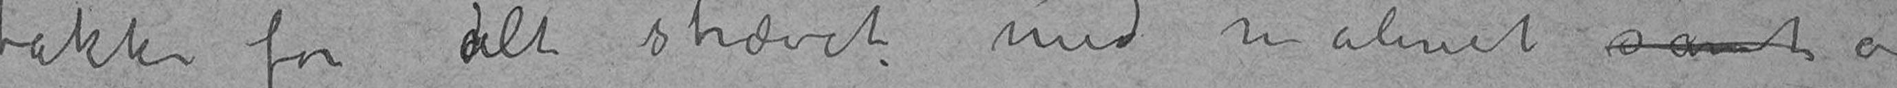takke for alt strævet med maleriet samt og
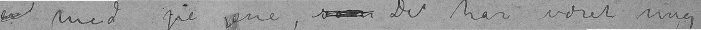med pengene, som Det har været mig
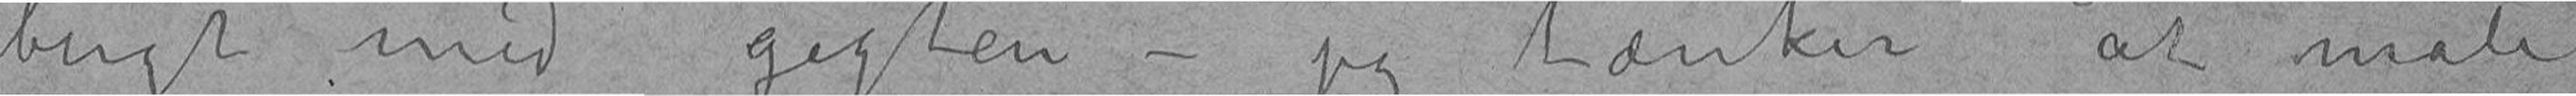bugt med gigten – jeg tænker at male
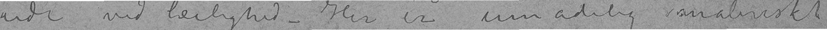ude ved leilighed – Her er umådelig maleriskt
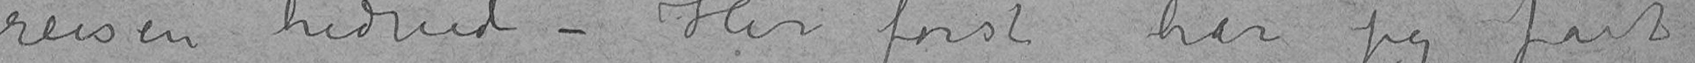reisen hidned – Her først har jeg fået
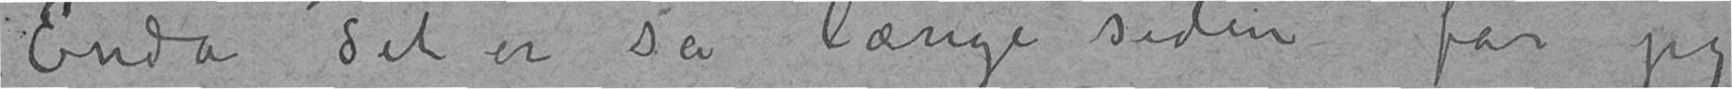Enda det er så længe siden får jeg
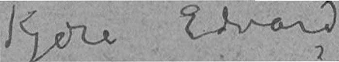Kjære Edvard
